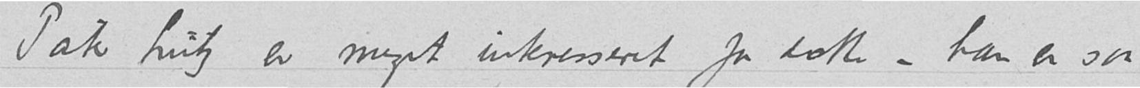Pater Lutz er meget interesseret for dette – han er saa
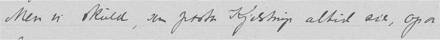Men vi skulde, som pastor Kjelstrup altid sier, ogsaa
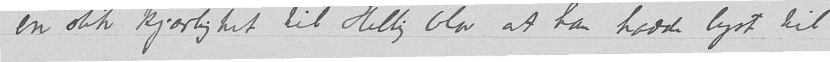en slik kjærlighet til Hellig Olav at han hadde lyst til
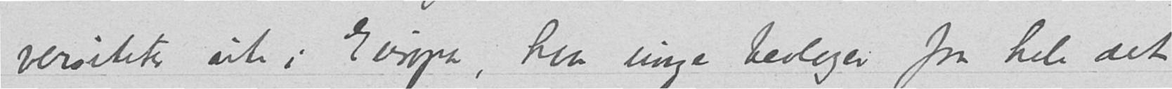versiteter ute i Europa, hvor unge teologer fra hele det
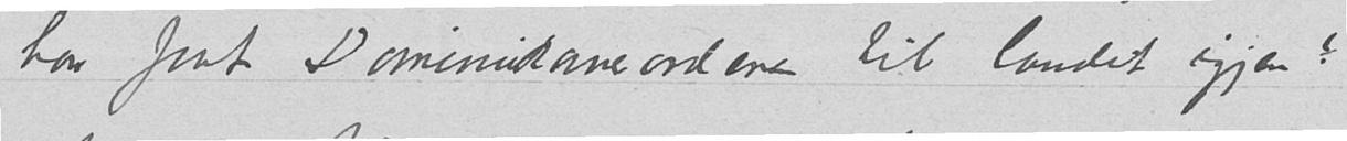har faat Dominikanerordenen til landet igjen?
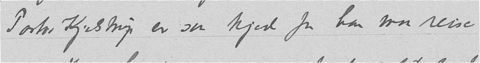Pastor Kjelstrup er saa kjed for han maa reise
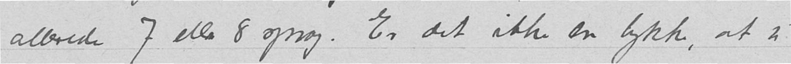allerede 7 eller 8 sprog. Er det ikke en lykke, at vi
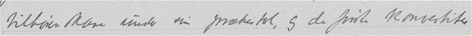tilhørerskare under sin prækestol, og de første konvertiter
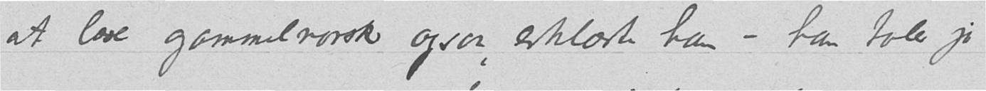at lære gammelnorsk ogsaa, erklærte han – han taler jo
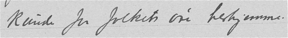kunde faa folkets øre herhjemme.
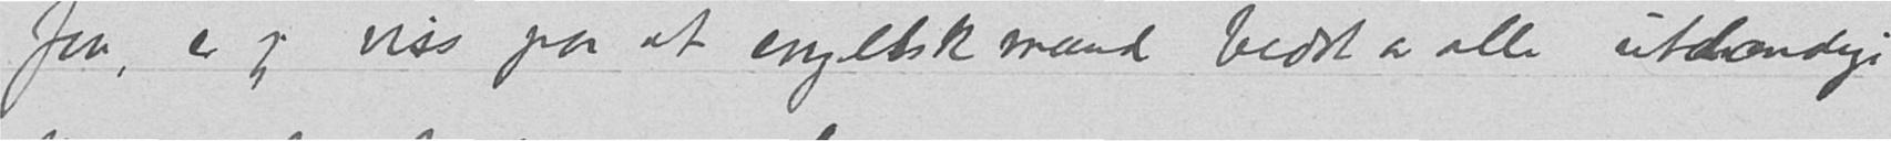faa, er jeg viss paa at engelskmænd bedst av alle utlændige
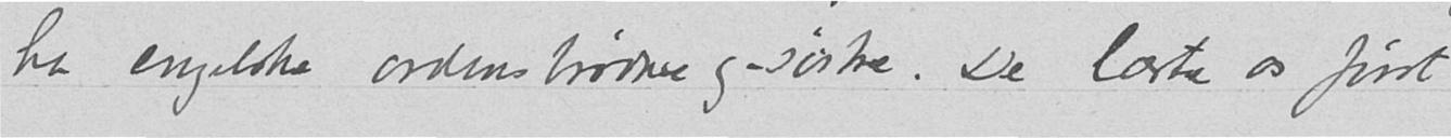ha engelske ordensbrødre og -søstre. De lærte os først
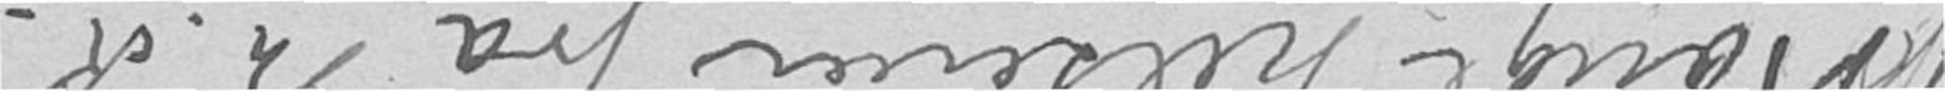Mange hilsener fra M.K.
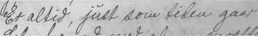Er altid, just som tiden gaar
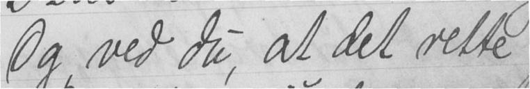Og ved du, at det rette
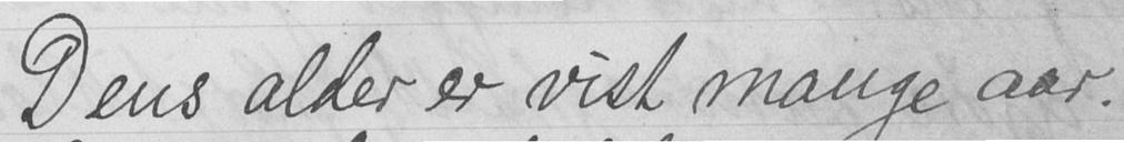Dens alder er vist mange aar.
